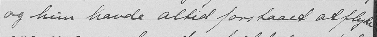og hun havde altid forstaaet at flyde
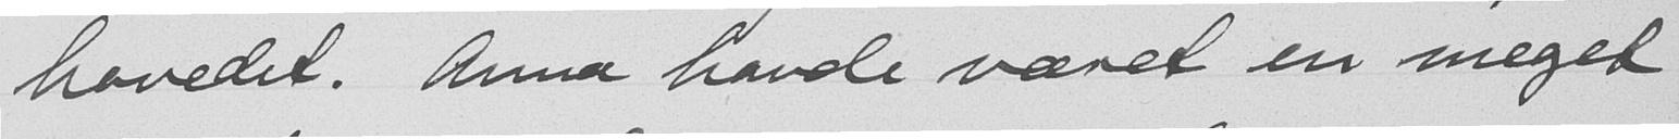hovedet. Anna havde været en meget
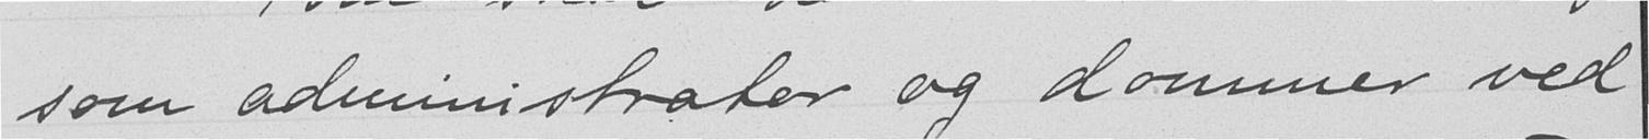som administrator og dommer ved
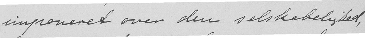imponeret over den selskabelighed,
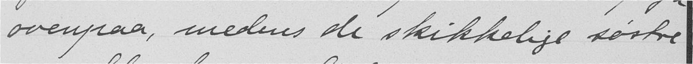ovenpaa, medens de skikkelige søstre
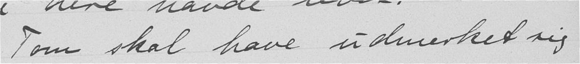Tom skal have udmerket sig
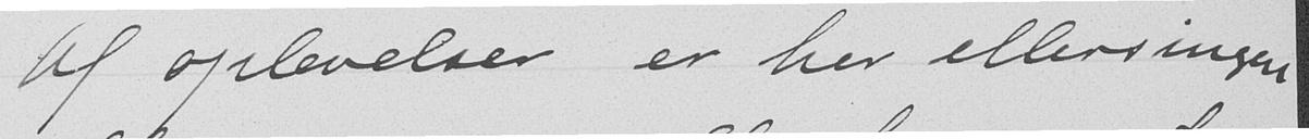Af oplevelser er her ellers ingen
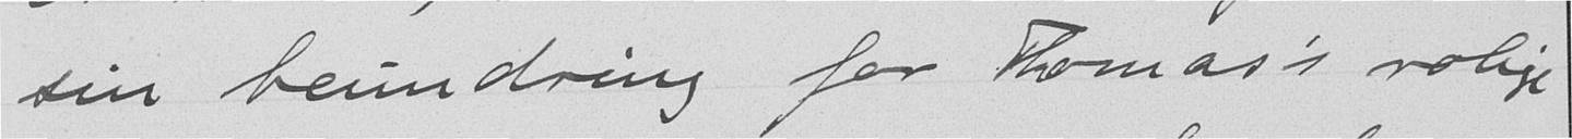sin beundring for Thomas's rolige
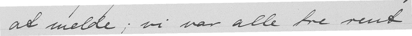at melde; vi var alle tre rent
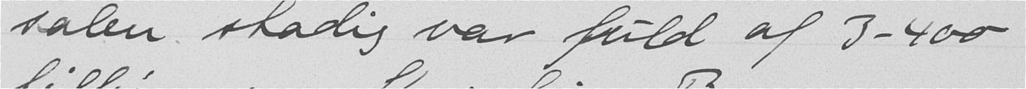salen stadig var fuld af 3-400
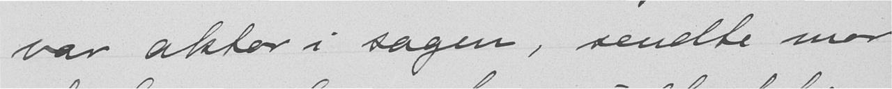var aktor i sagen, sendte mor
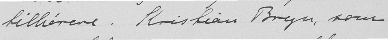tilhørere. Kristian Bryn, som
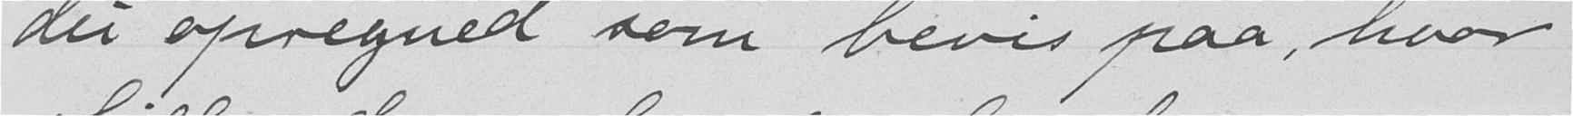du opregned som bevis paa, hvor
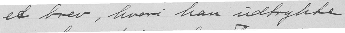et brev, hvori han udtrykte
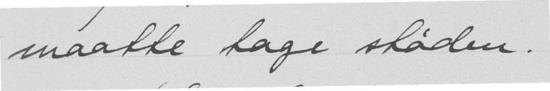maatte tage støden.
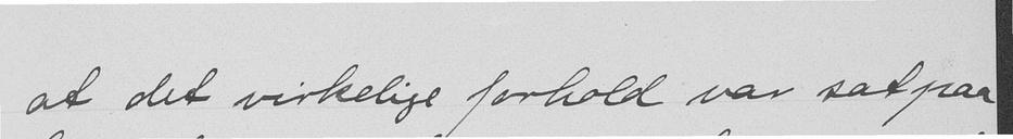at det virkelige forhold var sat paa
